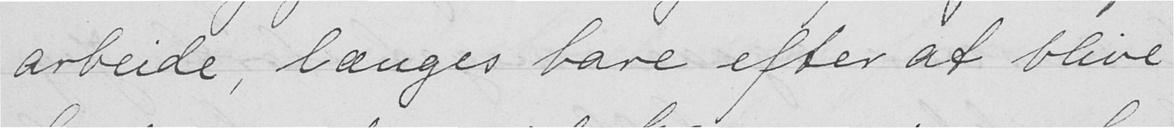arbeide, længes bare efter at blive
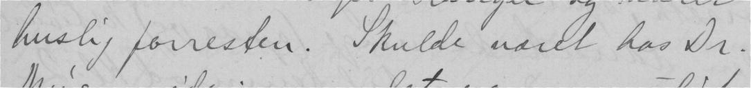huslig forresten. Skulde været hos Dr.
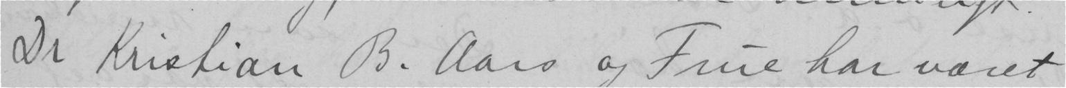Dr Kristian B. Aars og Frue har været
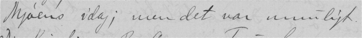Mjøens idag; men det var umuligt.
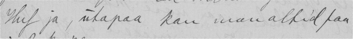Huf ja, utapaa, kan man altid faa
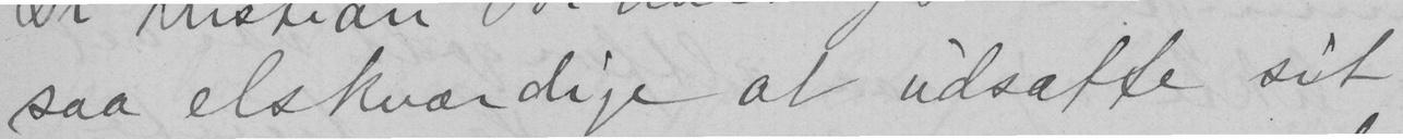saa elskværdige at udsætte sit
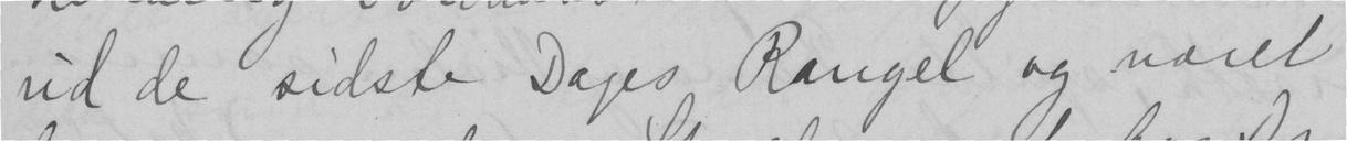ud de sidste Dages Rangel og været
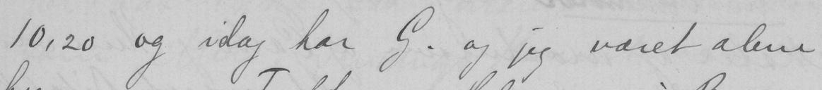10,20 og idag har G. og jeg været alene
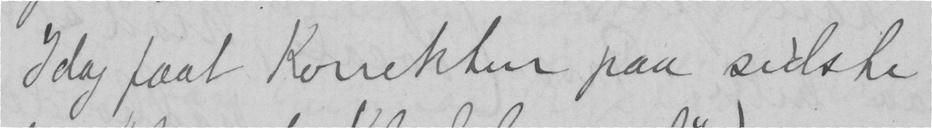Idag faat Korrektun paa sidste
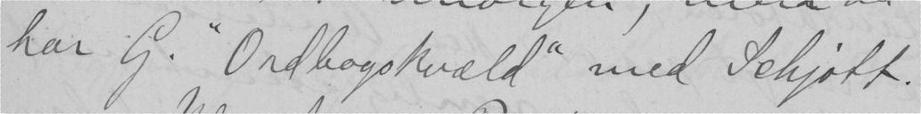har G. "Ordbogskvæld" med Schjøtt.
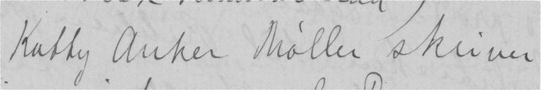Katty Anker Møller skriver
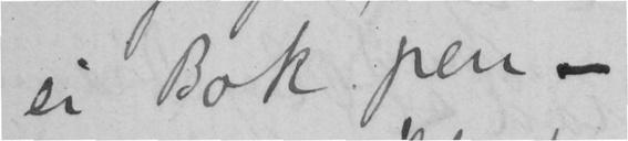ei Bok pen —
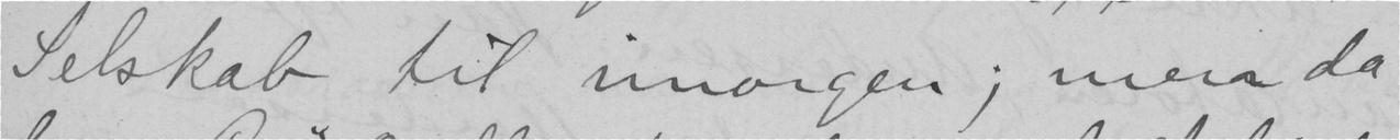Selskab til imorgen; men da
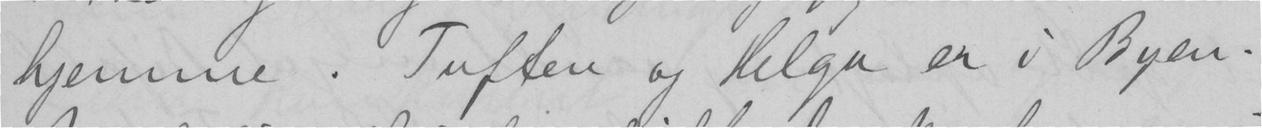hjemme. Tuften og Helga er i Byen.
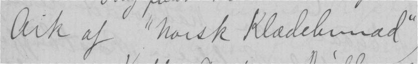ark af "norsk Klædebunad"
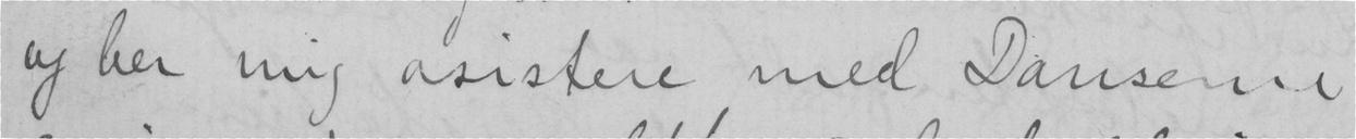og ber mig asistere med Danserne
